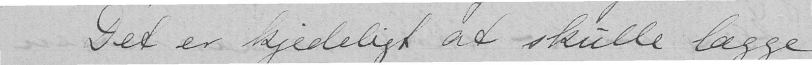Det er kjedeligt at skulle lægge
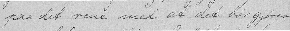paa det rene med at det bør gjøres,
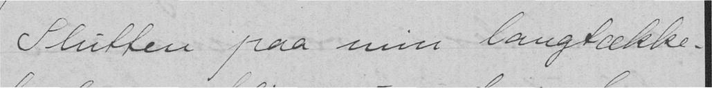Slutten paa min langtække-
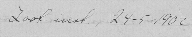Zoot. inst., 24-5-1902
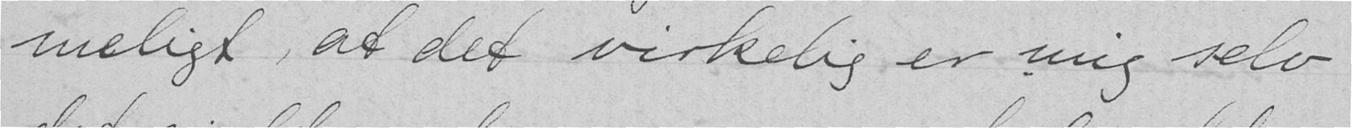meligt, at det virkelig er mig selv
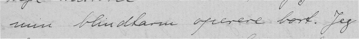min blindtarm operere bort. Jeg
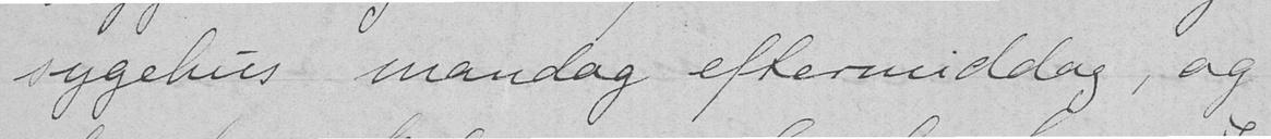sygehus mandag eftermiddag, og
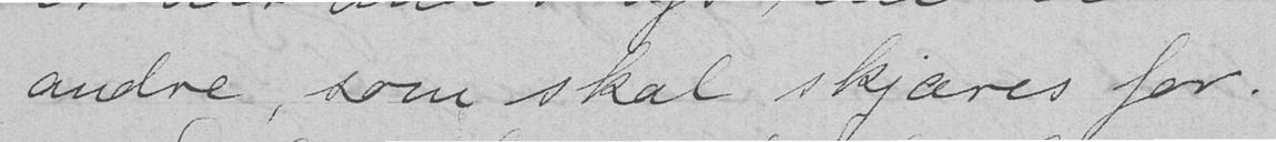andre, som skal skjæres for.
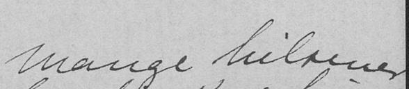Mange hilsener
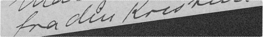fra din Kristine.
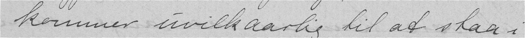kommer uvilkaarlig til at staa i
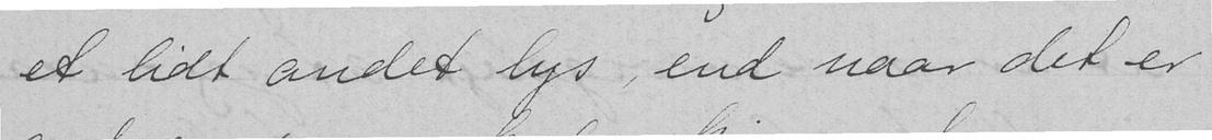et lidt andet lys, end naar det er
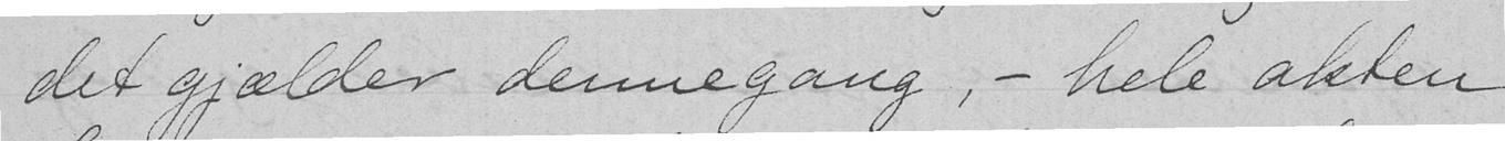det gjælder denne gang, - hele akten
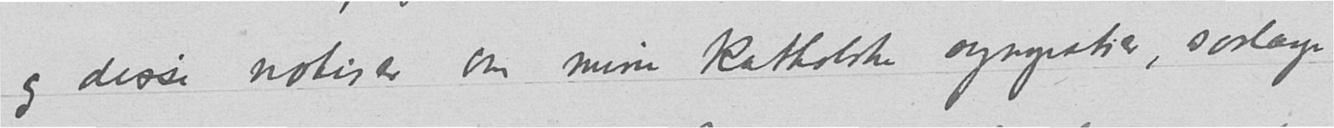og disse notiser om mine katholske sympatier, saalænge
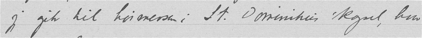jeg gik til høimessen i St. Dominikus' kapel, hvor
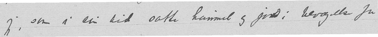jeg, som i sin tid satte himmel og jord i bevægelse for
